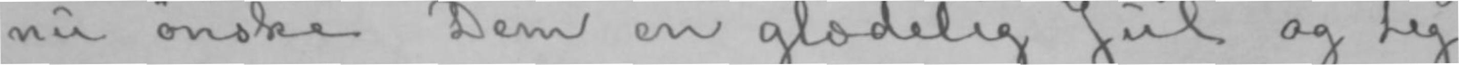nu ønske Dem en glædelig Jul og teg-
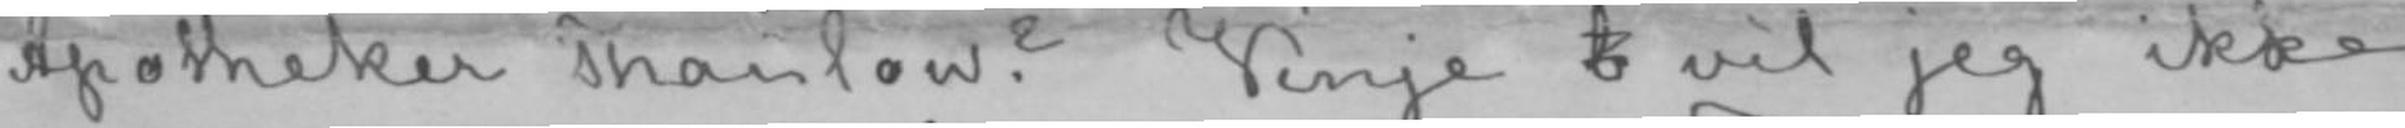Apotheker Thaulow? Vinje b vil jeg ikke
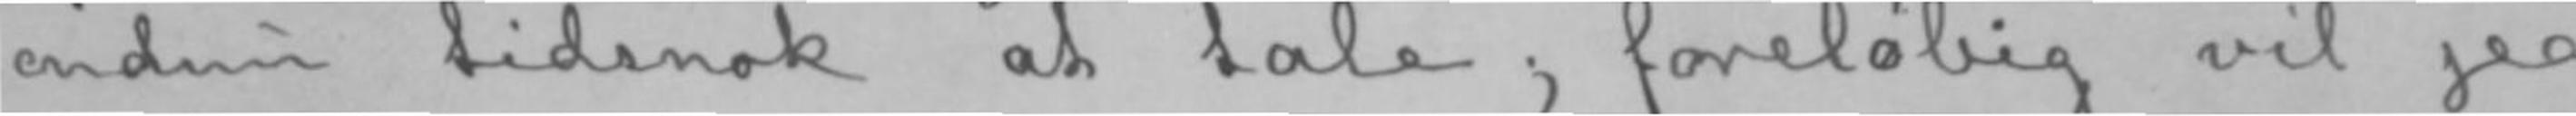endnu tidsnok at tale; foreløbig vil jeg
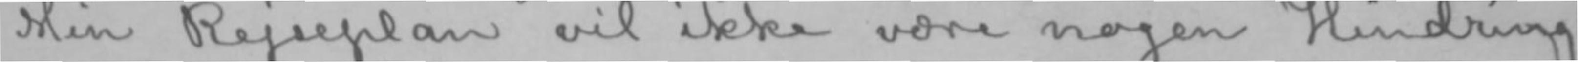Min Rejseplan vil ikke være nogen Hindring
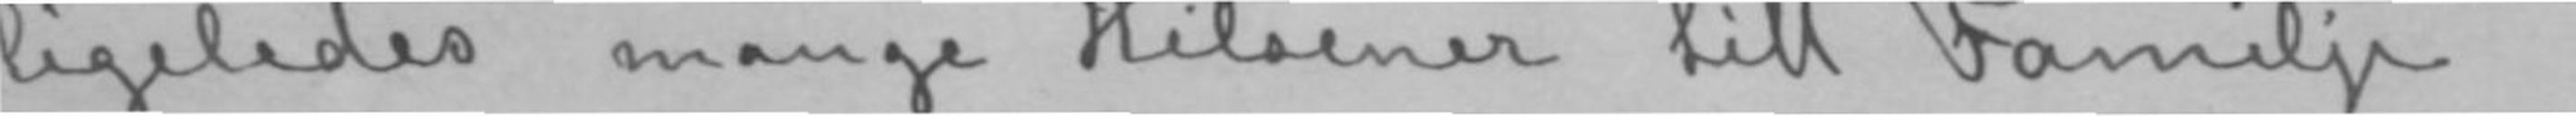ligeledes mange Hilsener till Familje og
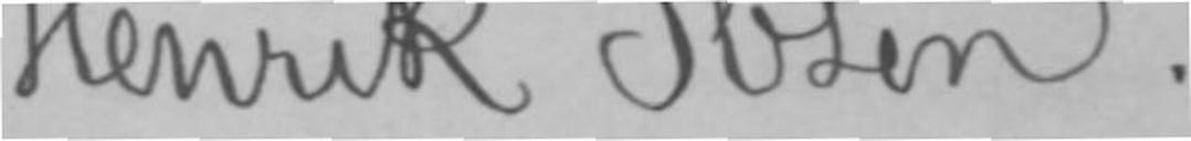Henrik Ibsen.
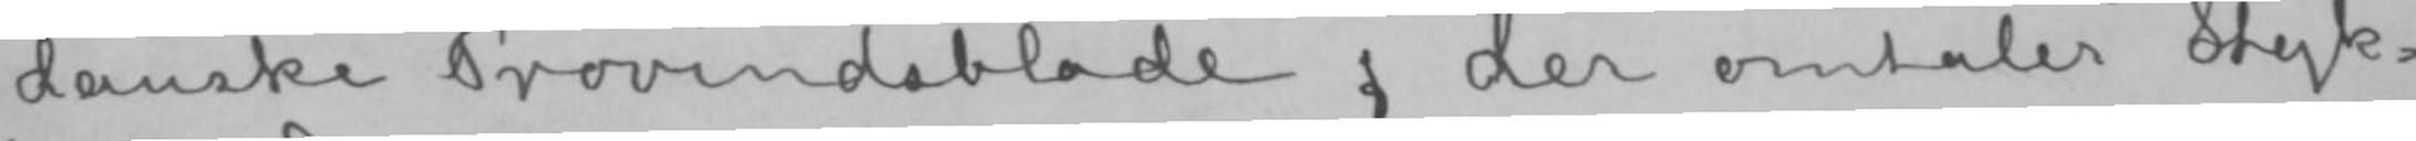danske Provindsblade, der omtaler Styk-
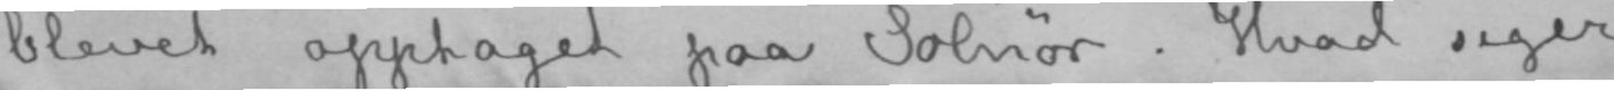blevet opptaget paa Solnør. Hvad siger
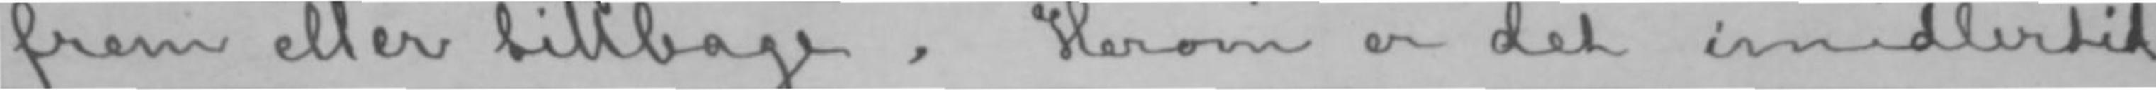frem eller tillbage. Herom er det imidlertid
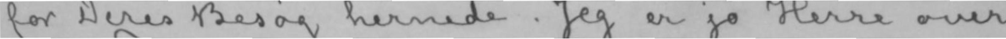for Deres Besøg hernede. Jeg er jo Herre over
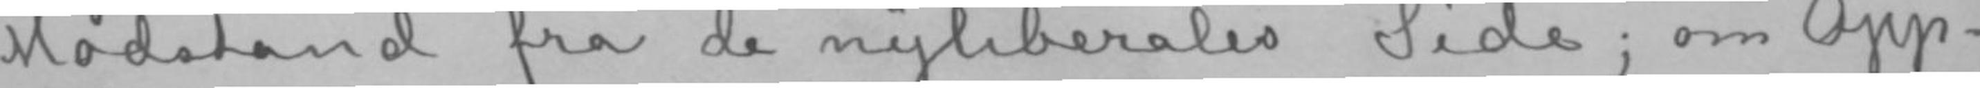Modstand fra de nyliberales Side; om Opp-
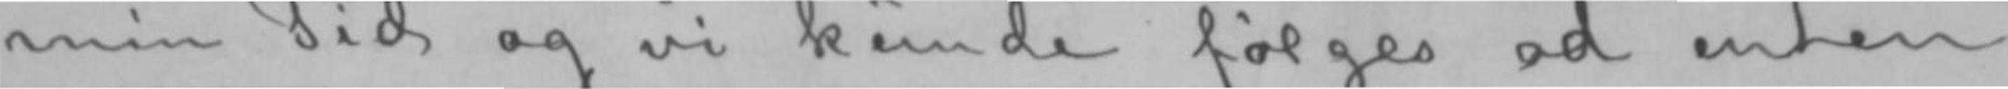min Tid og vi kunde følges ad enten
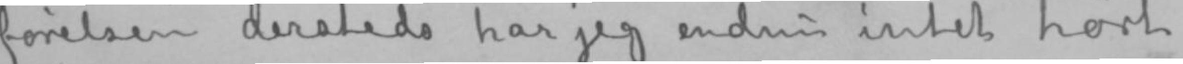førelsen dersteds har jeg endnu intet hørt.
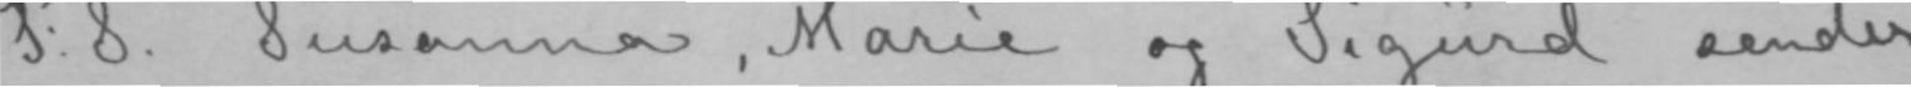P: S. Susanna, Marie og Sigurd sender
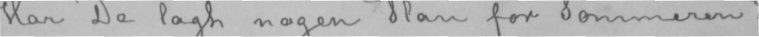Har De lagt nogen Plan for Sommeren?
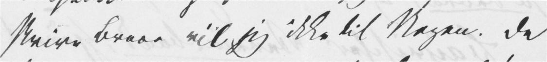skrive Breve vil jeg ikke til Nogen. De
